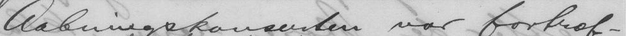Aabningskonserten var fortræf-
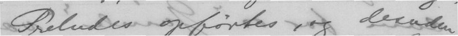Preludes opførtes, og desudenen
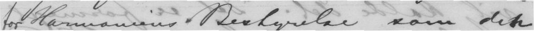for Harmoniens Bestyrelse som den
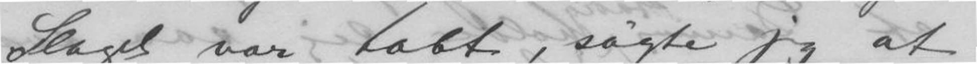Slaget var tabt, søgte jeg at
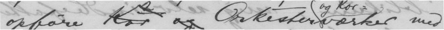opføre Kor og Orkester værker med
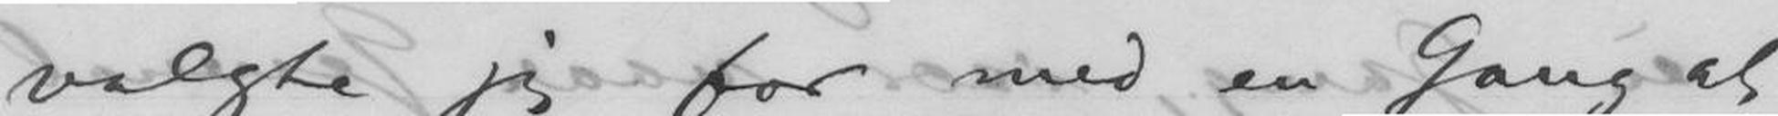valgte jeg for med en Gang at
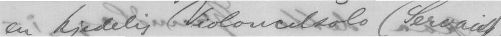kjedelig Violoncelsolo (Servait)
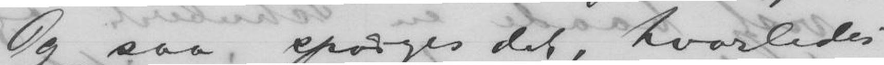Og saa spørges det, hvorledes
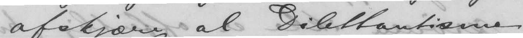afskjære al Dilettantisme
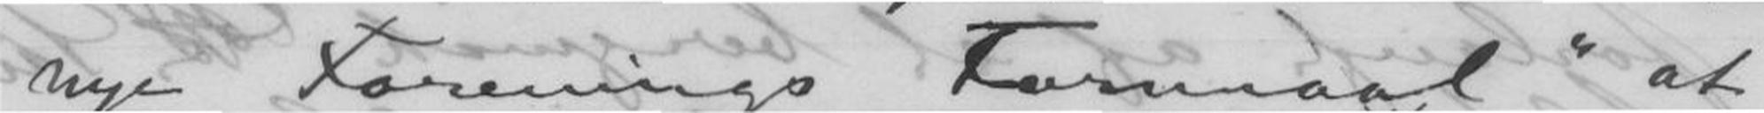nye Forenings Formaal "at
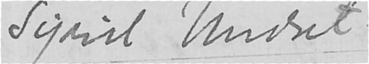Sigrid Undset.
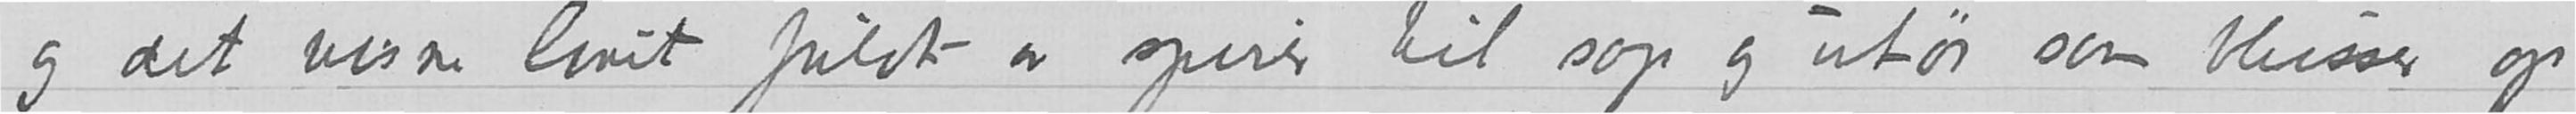og det visne løvet fuldt av spirer til sop og <utøir> som blusser op
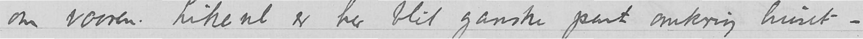om vaaren. Likevel er her blit ganske pent omkring huset –
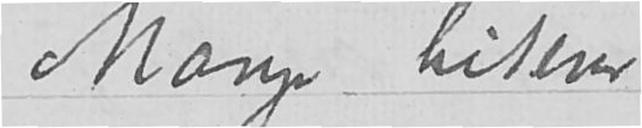Mange hilsener
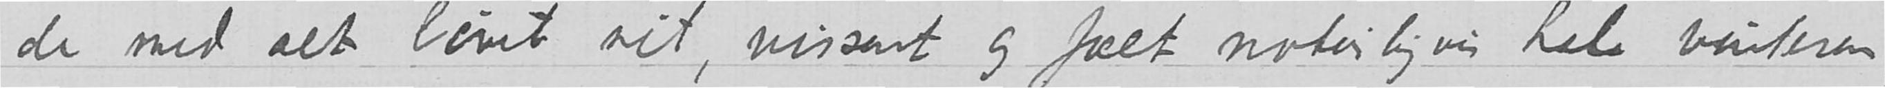de med alt løvet sit, vissent og fælt naturligvis hele vinteren,
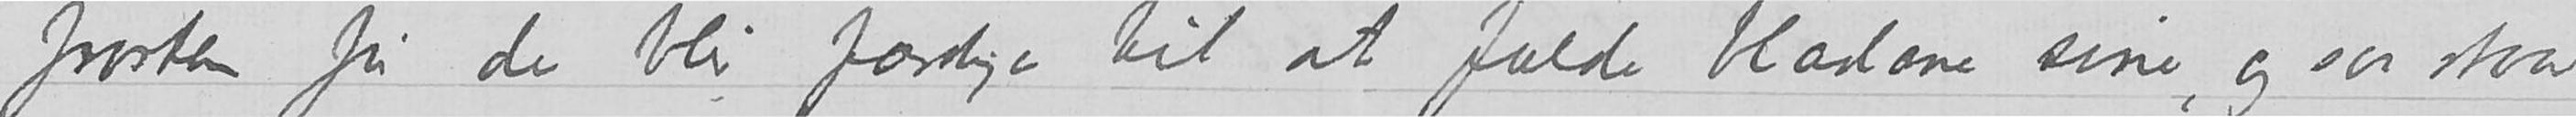frosten før de blir færdige til at fælde bladene sine, og saa staar
